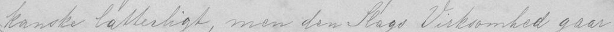kanske latterligt, men den Slags Virksomhed gaar
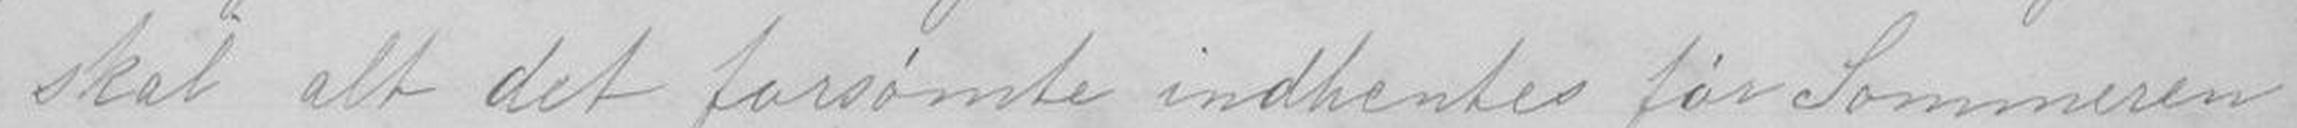skal alt det forsømte indhendes før Sommeren
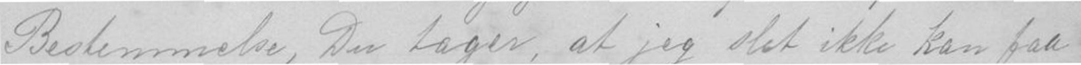Bestemmelse, D tager, at jeg slet ikke kan faa
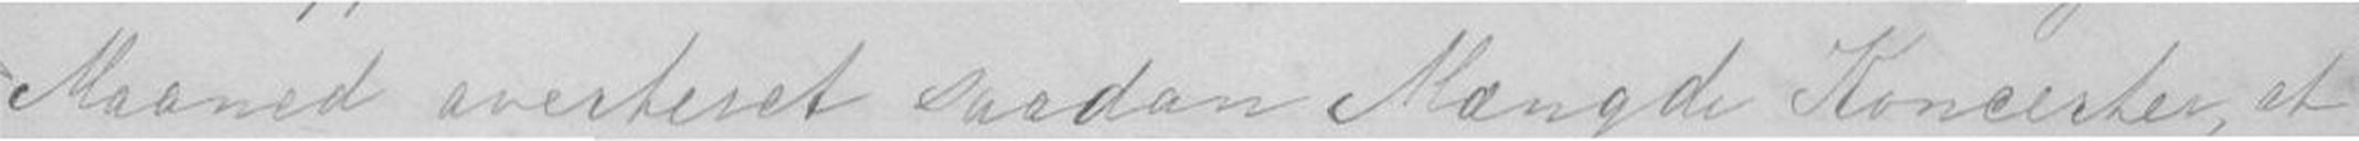Maaned overdrevet saadan Mængde Koncerter, at
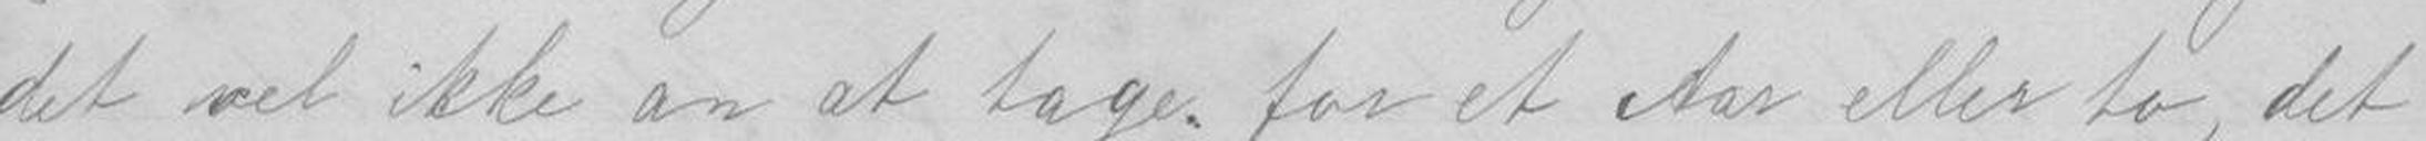det vel ikke an at tage for et tas eller to, det
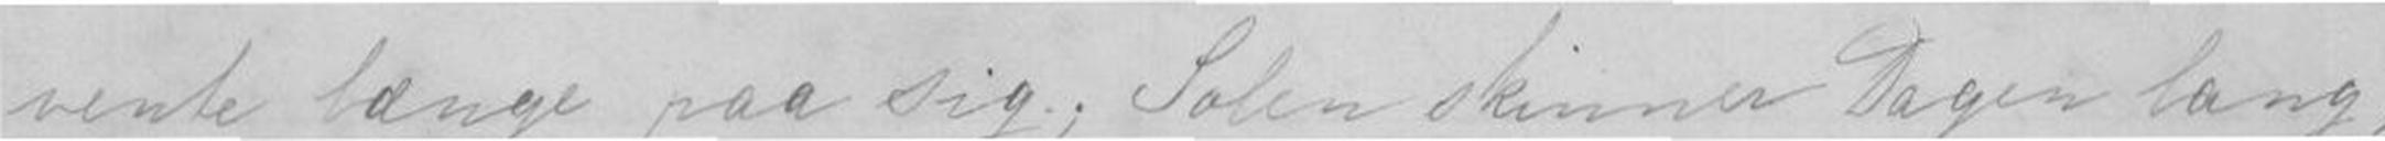vente længe paa sig; Solen skinner Dagen lang,
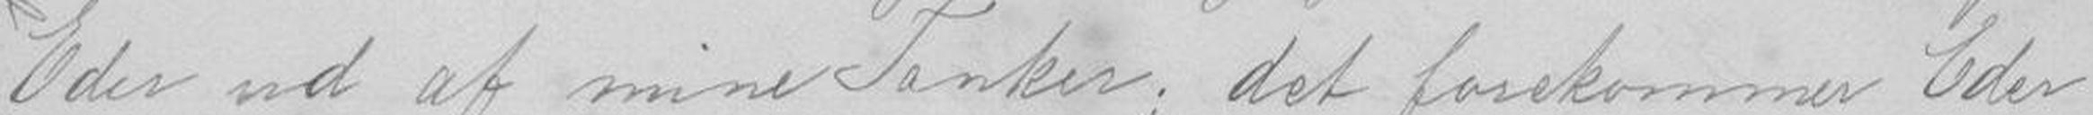Eder ud af mine Tanker; det forekommer Eder
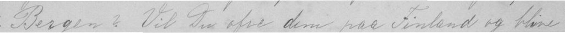Bergen? Vil Du ofre dem paa Finland og blive
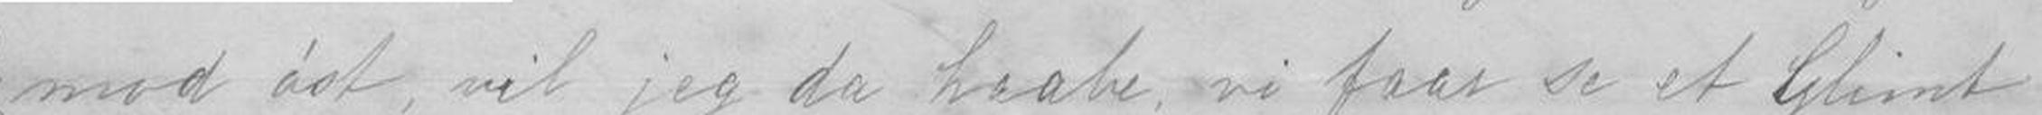mod øst, vil jeg da haabe vi faar se et Glimt
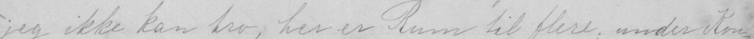jeg ikke kan tro, her er Rum til flere; under Kon-
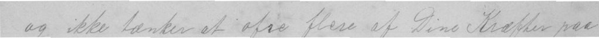og ikke tænker at ofre flere af Dine Kræfter paa
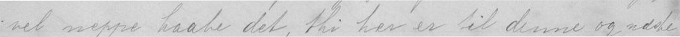vel neppe haabe det, thi her er til denne og næste
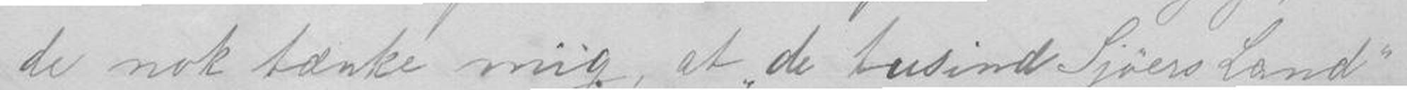de nok tænke mig at de tusind Sjøers Land
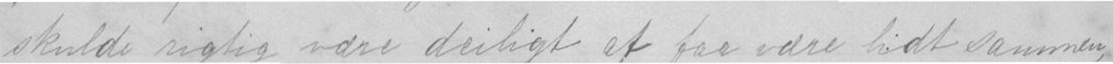skulde rigtig være deiligt af faa være lidt sammen,
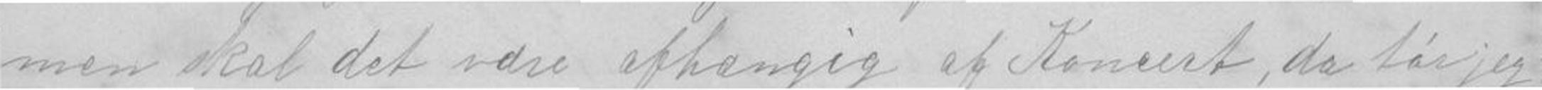men skal det være afhengig af Koncert, da får jeg
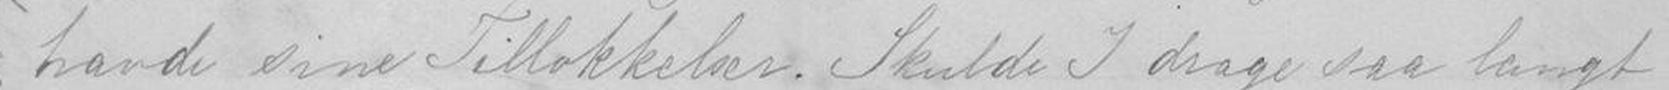havde sine Tillokkelser. Skulde I drage saa langt
